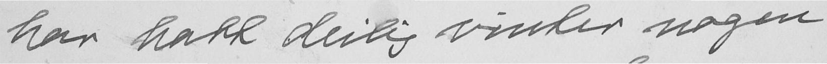har hatt deilig vinter nogen
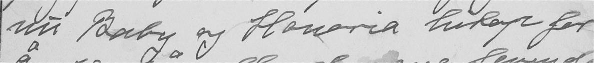nu Baby og Honoria hitop for
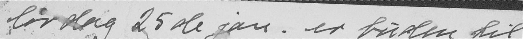lørdag 25de jan. er buden til
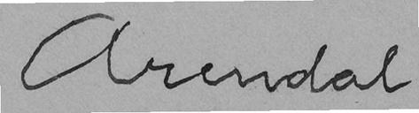Arendal
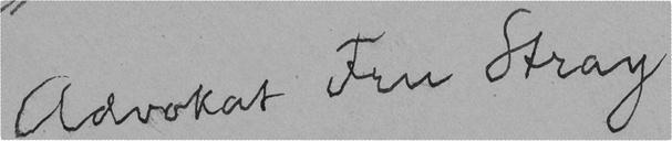Advokat Fru Stray
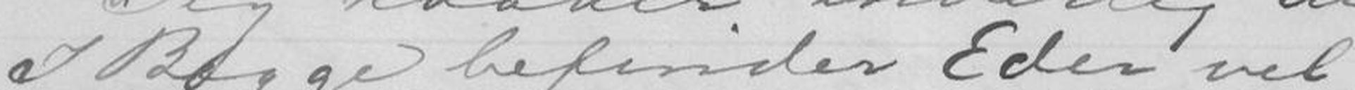I Begge befinder Eder vel,
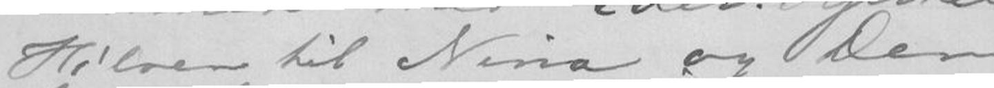Hilsen til Nina og Dem
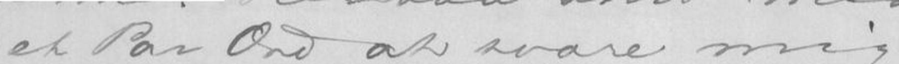et Par Ord at svare mig
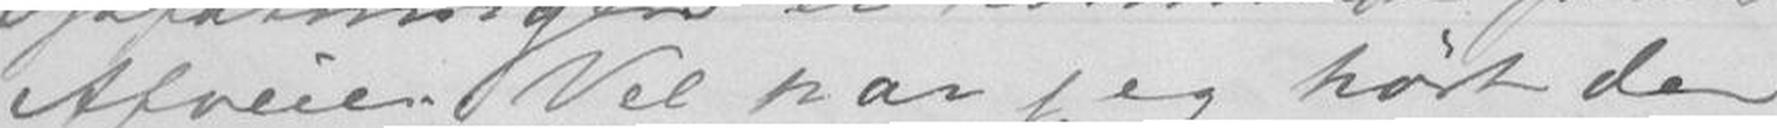Afveie. Vel har jeg hørt den
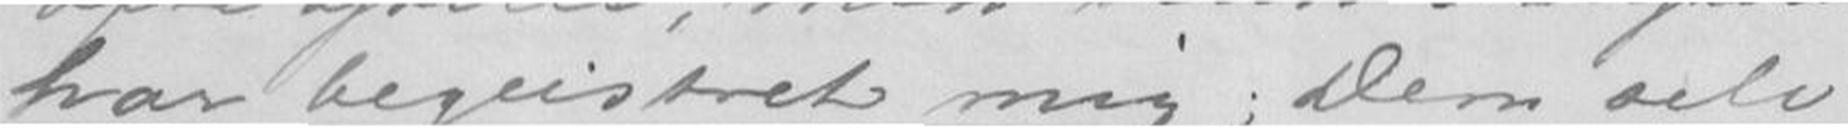har begeistret mig; Dem selv
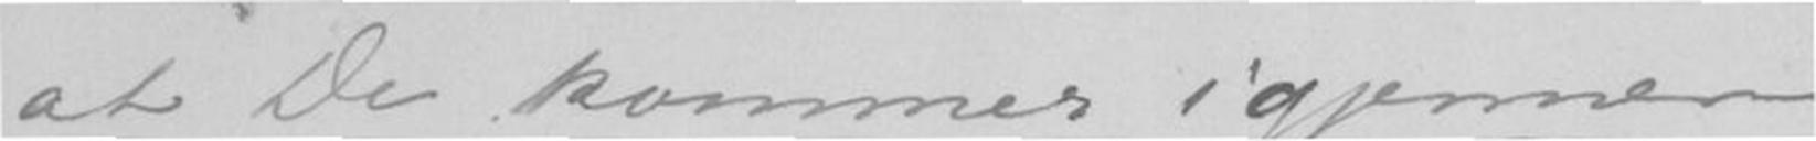at De kommer igjennem
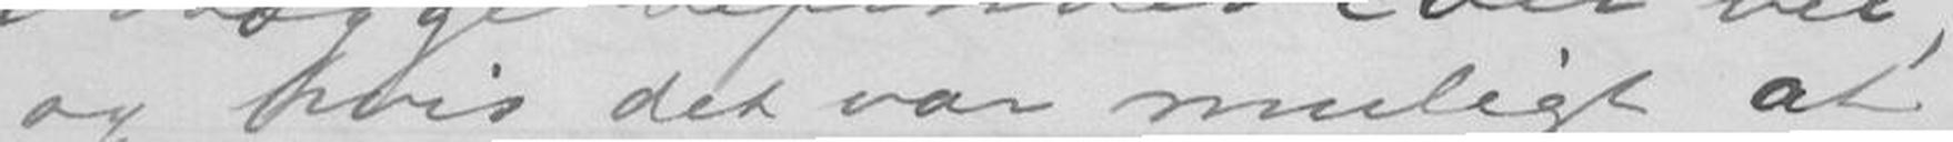og hvis det var muligt at
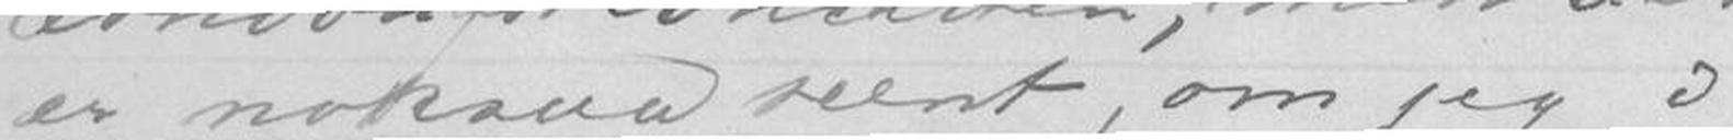er noksaa seent, om jeg i
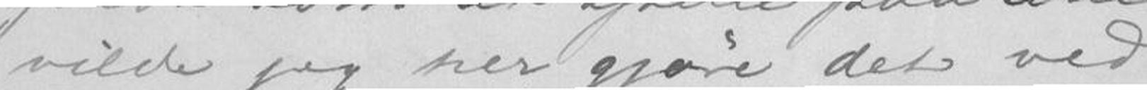vilde jeg her gjøre det ved
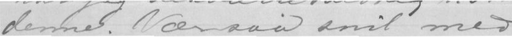denne. Vær saa snil med
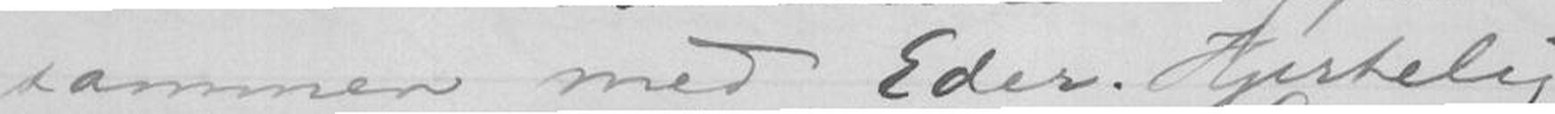sammen med Eder. Hjertelig
